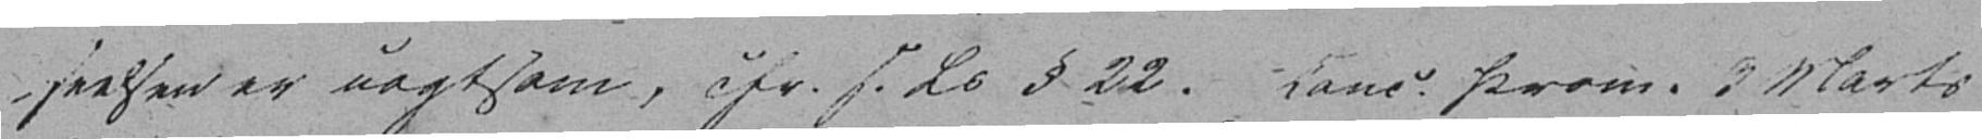seelsen er uagtsom, cfr. f. Ls § 22. Kanc. Prom. 3 Marts
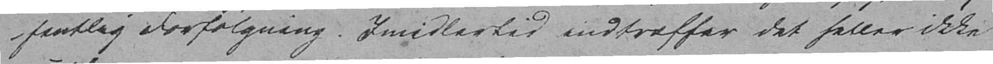fentlig Forfølgning. Imidlertid indtræffer det heller ikke
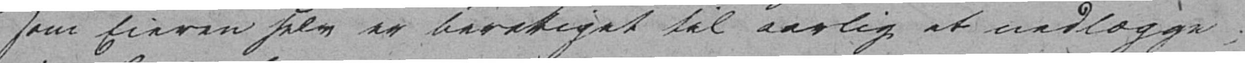som Eieren selv er berettiget til aarlig et nedlægge;
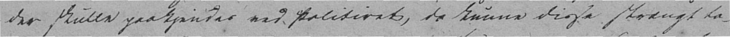der skulle paakjendes ved Politiret, da kunne disse strængt ta-
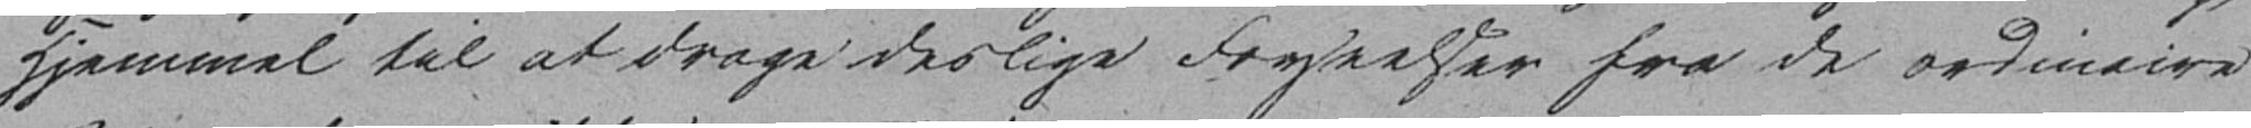Hjemmel til at drage deslige Forseelser fra de ordinaire
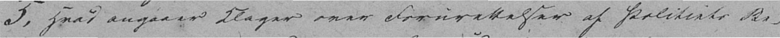5, Hvad angaaer Klager over Forurettelser af Politiets Be-
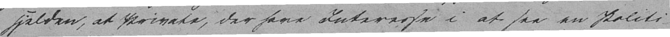sjelden, at Private, der have Interesse i at see en Politi-
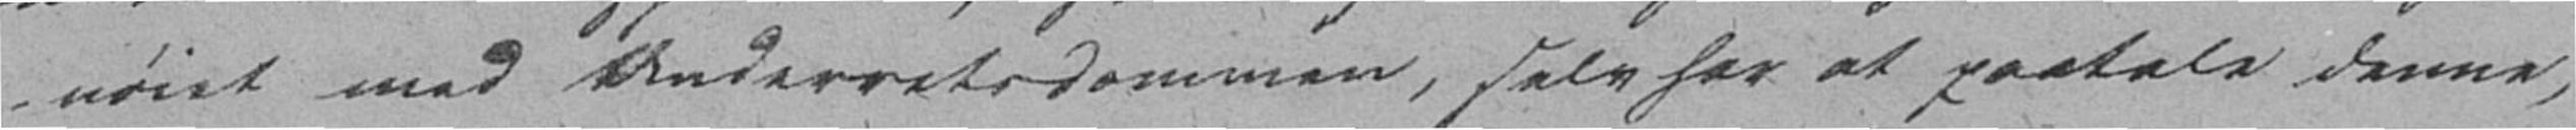nøiet med Underretsdommen, selv har at paatale denne,
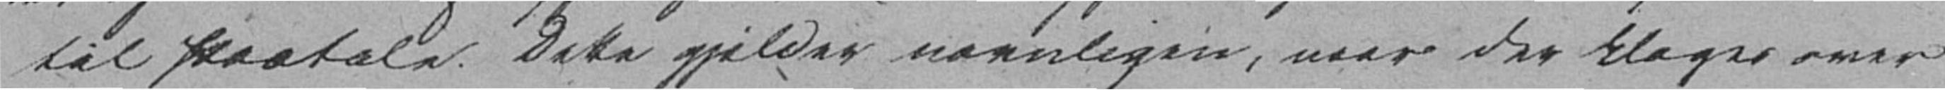til Paatale. Dette gjelder navnligen, naar der klages over
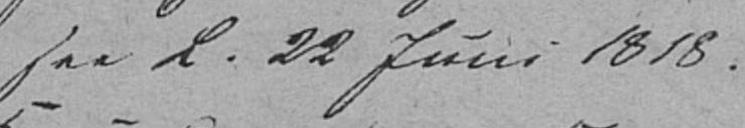see L. 22 Juni 1818.
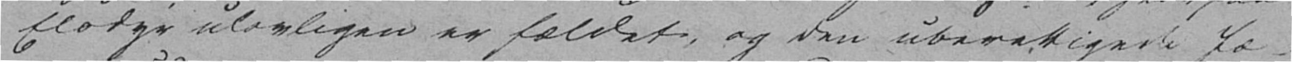Elsdyr ulovligen er fældet, og den uberettigede Jæ-
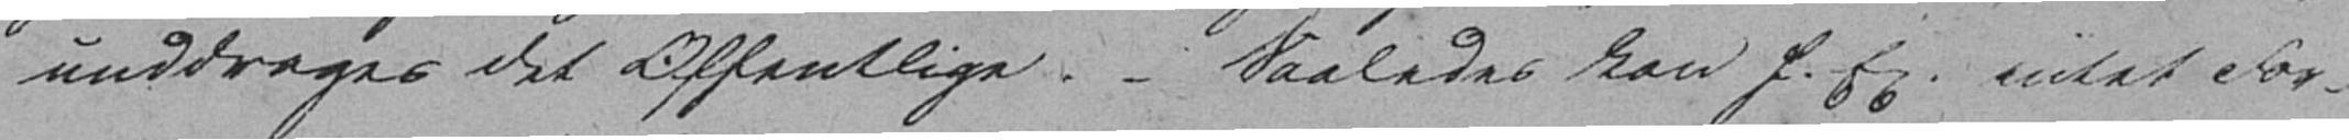unddrages det Offentlige. – Saaledes kan f. Ex. intet For-
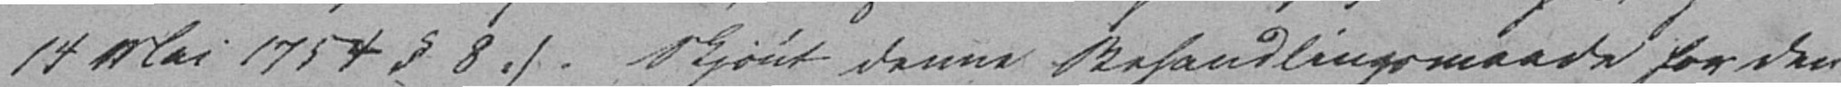14 Mai 1754 § 8 :). Skjønt denne Behandlingsmaade for den
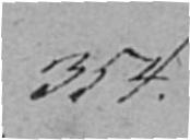354.
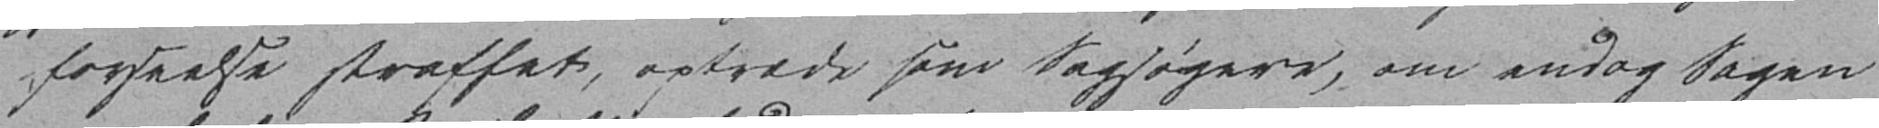forseelse straffet, optræde som Sagsøgere, om endog Sagen
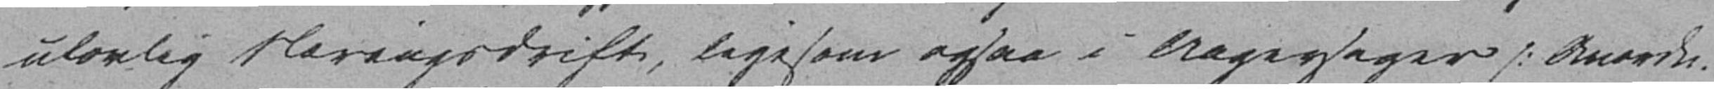ulovlig Næringsdrift, ligesom ogsaa i Aagersager (: Bnævd.
